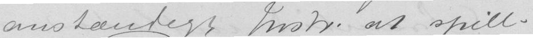anstændigt Instr. at spille
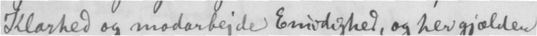Klarhed og modarbejde Ensidighed og her gjælder
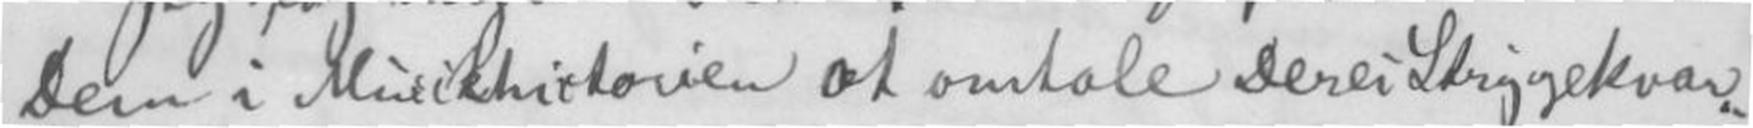Dem i Musikhistorien at omtale Deres Strygekvar-
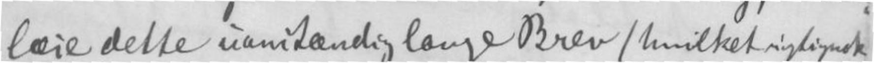læse dette uanstændig lange Brev (hvilket rigtignok
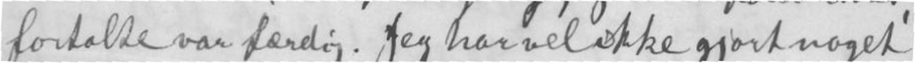fortalte var færdig, Jeg har vel ikke gjort noget
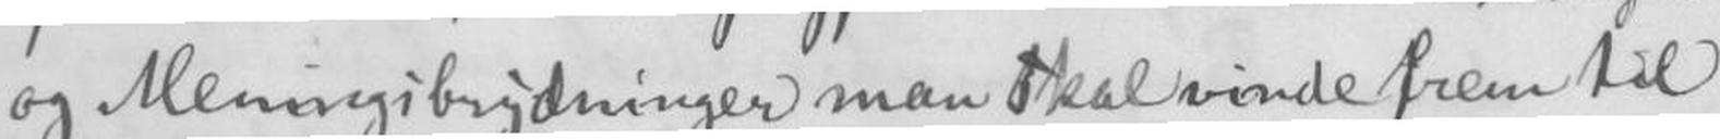og Meningsbrydninger man skal vinde frem til
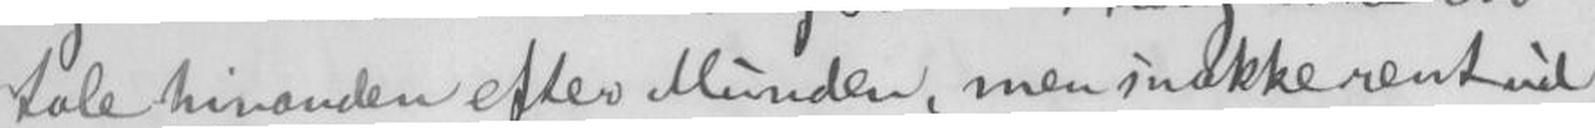tale hinanden efter Munden, men snakke rent ud
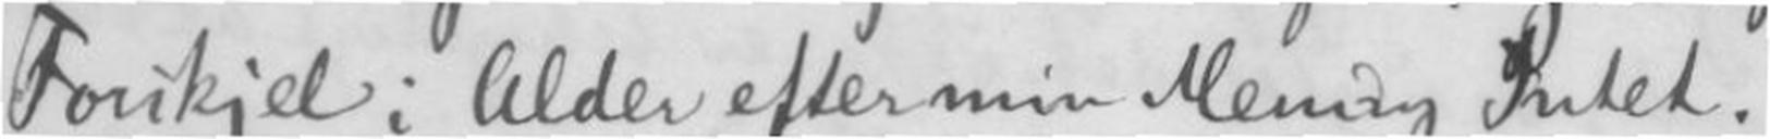Forskjel i Alder efter min Mening Intet. -
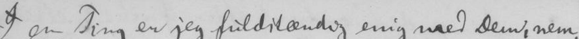I en Ting er jeg fuldstændig enig med Dem, nem-
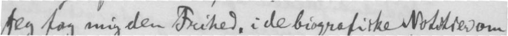Jeg tog mig den Frihed, i de biografiske Notitser om
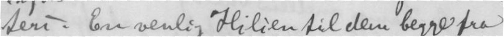ters. En venlig Hilsen til dem begge fra
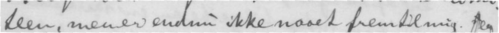teen, man er endnu ikke naaet frem til mig. Jeg
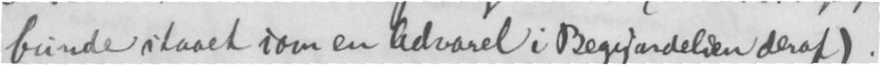burde staaet som en Advarsel i Begyndelsen deraf):
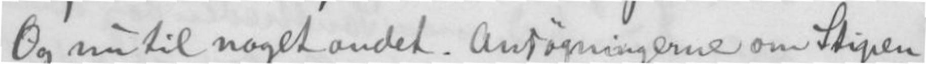Og nu til noget andet. Ansøgningen om Stipen-
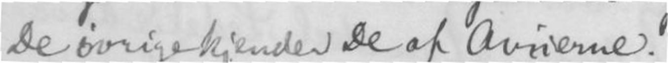De øvrige kjender De af Aviserne.
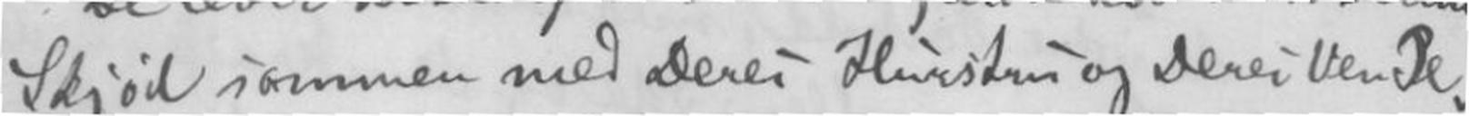Skjød sammen med Deres Hustru og Deres Ven Pe-
